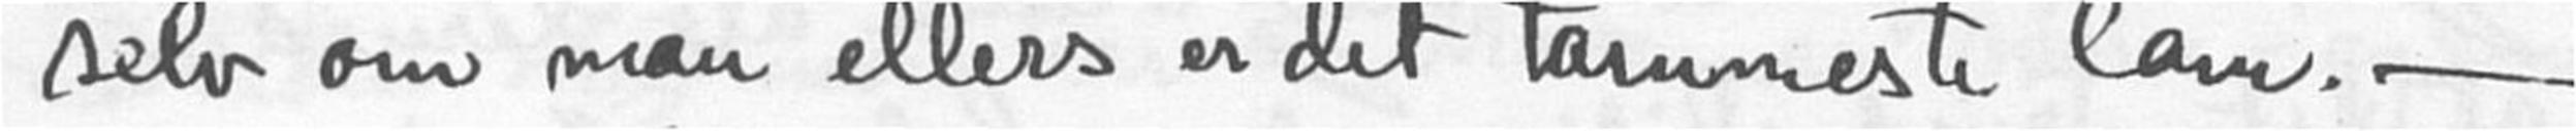selv om man ellers er det tammeste lam. -
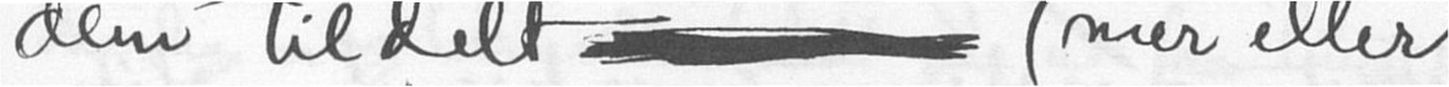dem tildelt - (mer eller
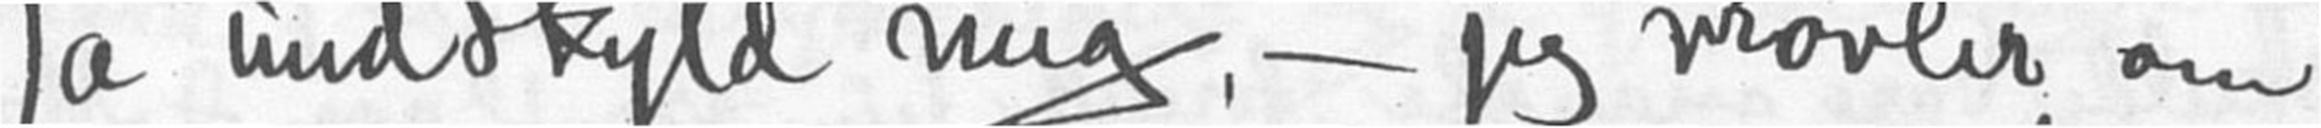Ja unnskyld mig, - jeg vrøvler om
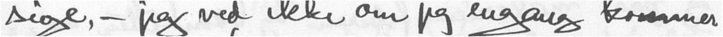sige, - jeg ved ikke om jeg engang kommer
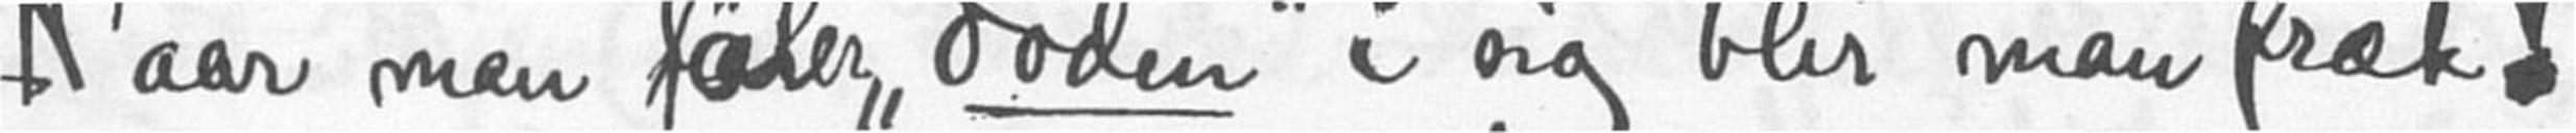Naar man føler "døden" i sig blir man fræk!
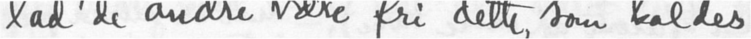Lad de andre være fri dette, som kaldes
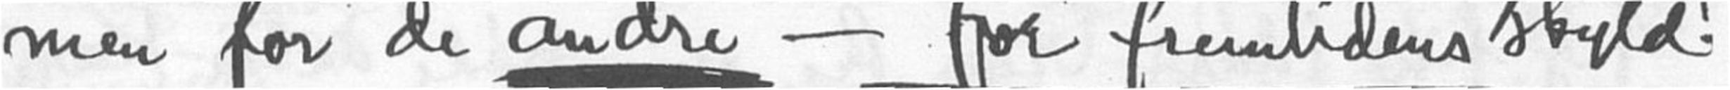men for de andre - for fremtidens skyld!
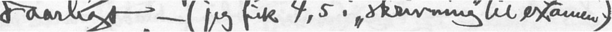daarligt - (jeg fik 4,5 i "skrivning" til examen).
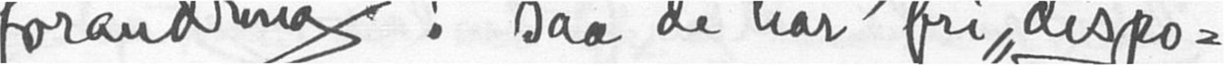forandring ! Saa de har fri "dispo-
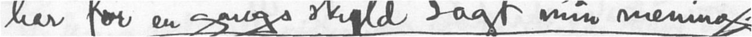har for en gangs skyld sagt min mening.
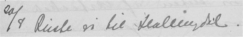20/8 Reiste vi til Hallingdal.
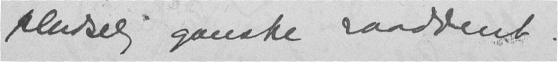pludselig ganske raaddent.
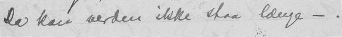Da kan verden ikke staa længe - .
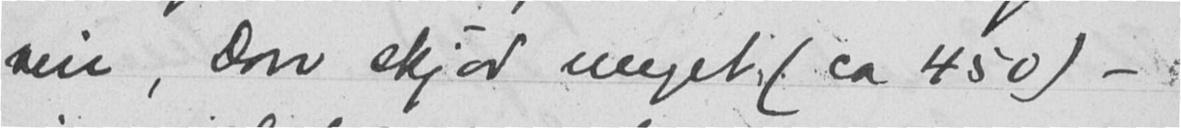veir, Don skjød meget (ca 450) -
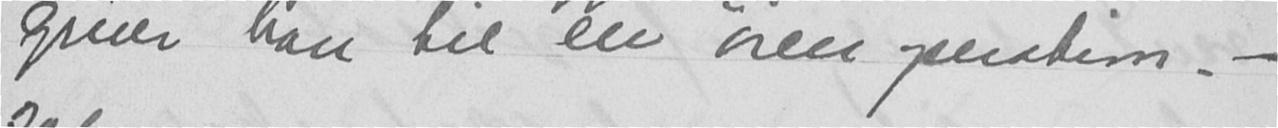gruer han til en øienoperation. -
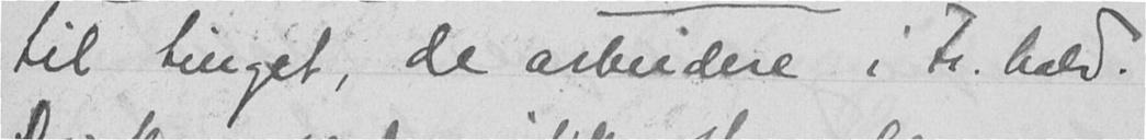til tinget, de arbeidere i Fr. hald.
